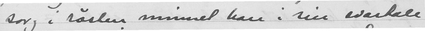sorg i røsten minnet han i sin svartale
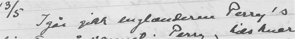13/5 Igår gikk englenderen Perry's
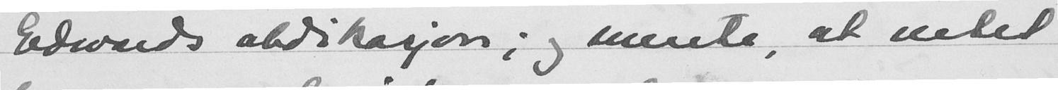Edvards abdikasjon; og mente, at intet
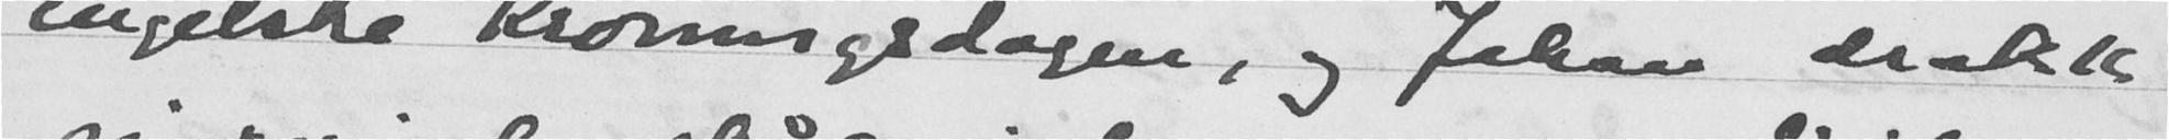engelske Kroningsdagen, og Johan drakk
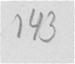143
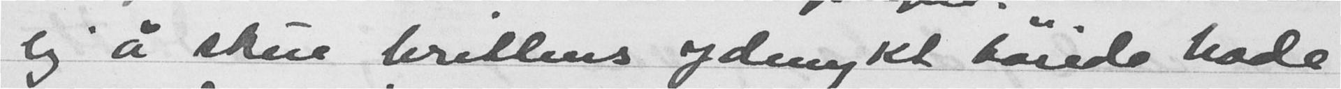lig å skue brittens ydmykt bøiede hode
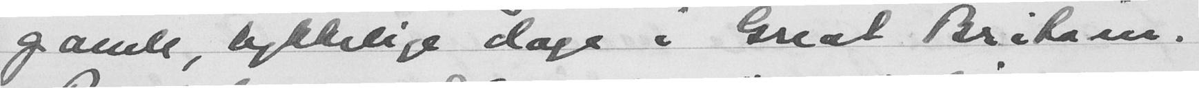gamle, lykkelige dage i Great Britain.
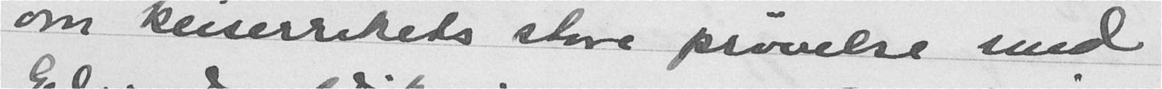om keiserrikets store prøvelse med
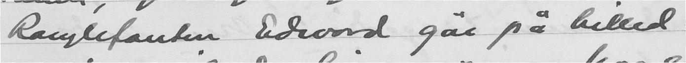Ranglefanten Edvard går på billed
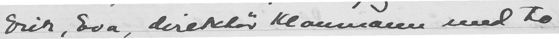Erik, Eva, direktør Klanmann med to
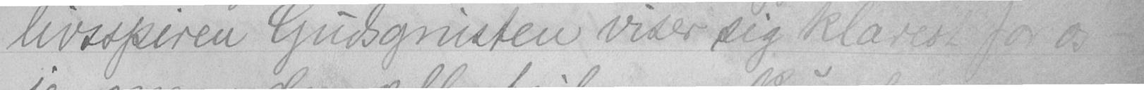livsspiren Gudsgnisten viser sig klarest for os -
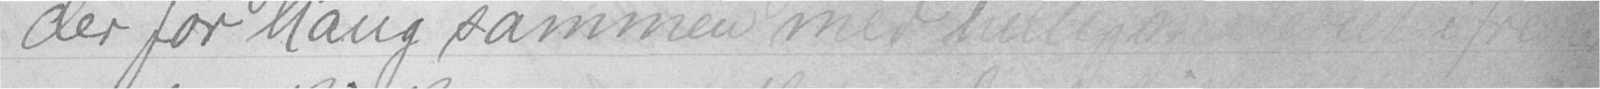der før hang sammen med hellig
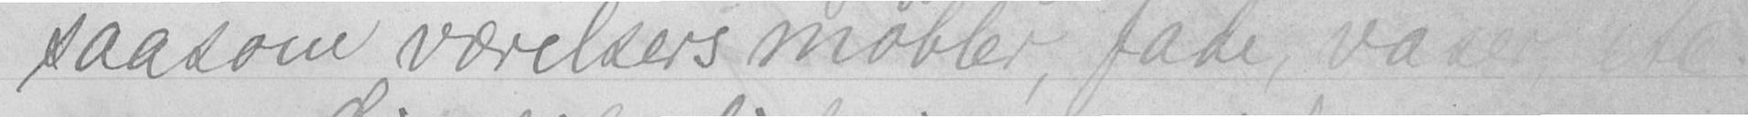saasom værelsers møbler, fade, vaser etc.
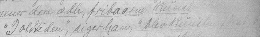"I oldtiden", siger han, "blev kunsten først og
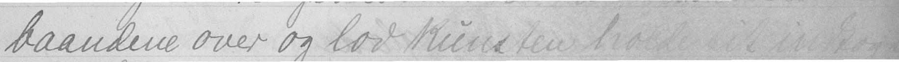baandene over og lod kunsten holde sit indtog i
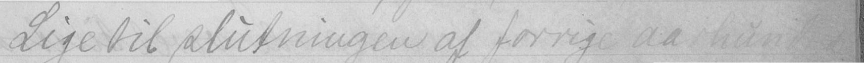Lige til slutningen af forrige aarhundrede
